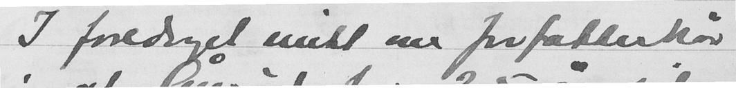I foredraget mitt om forfatterkår
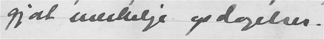gjort merkelige opdagelser.
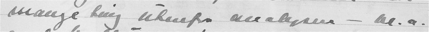mange ting utenfor analysen - bl. a.
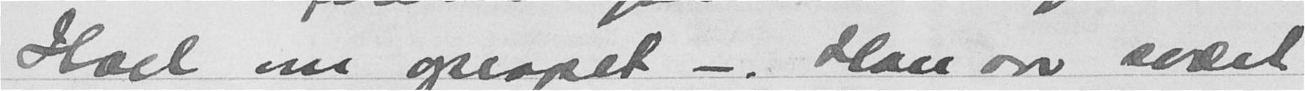Hoel om opropet -. Han var svært
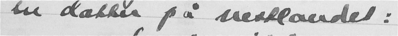en datter på vestlandet!
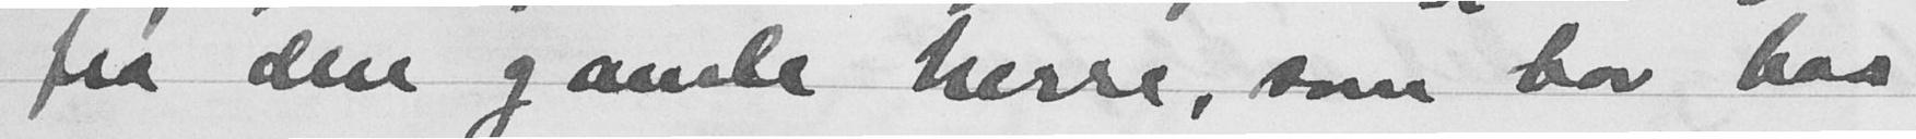fra den gamle herre, som bor hos
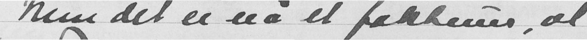Men det er nå et faktum, at
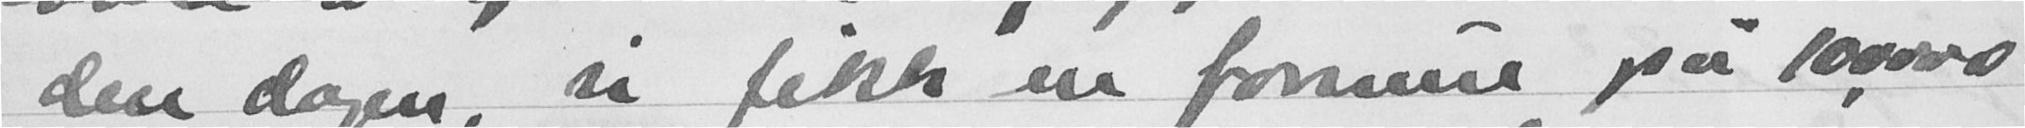den dagen, vi fikk en formue på 100000
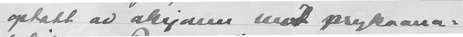optatt av aksjonen mot psykoana-
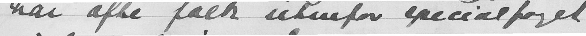har ofte folk utenfor specialfaget
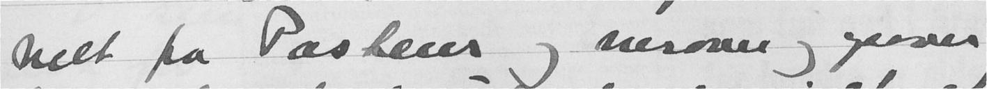helt fra Pasteus og nerøver og opover
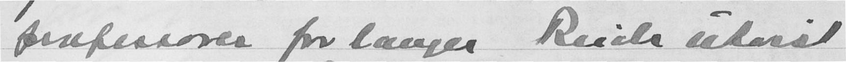professorer forlanger Reich utvist
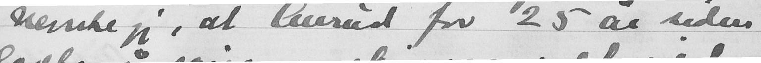nevnte jeg, at Ånerud for 25 år siden
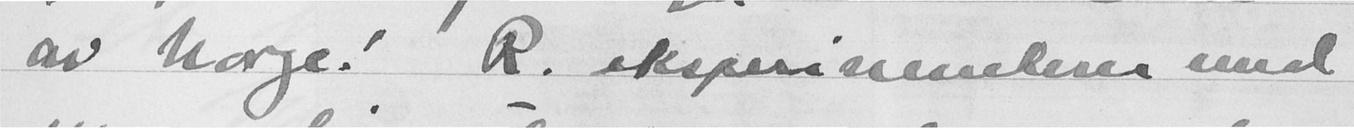av Norge. R. ekspirementerer med
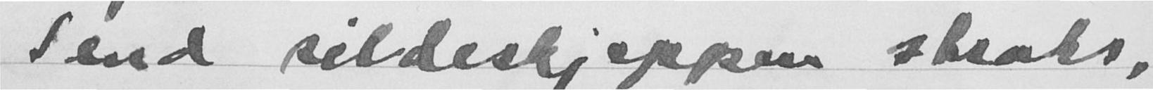Send sildeskjeppen straks,
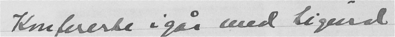Konfererte igår med Sigurd
